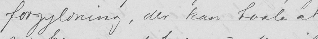forgyldning, der kan taale at
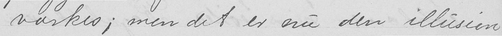vaskes; men det er nu den illusion
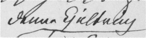denne Kjeltring
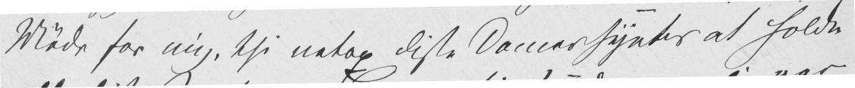Møde for mig, thi netop disse Damer syntes at holde
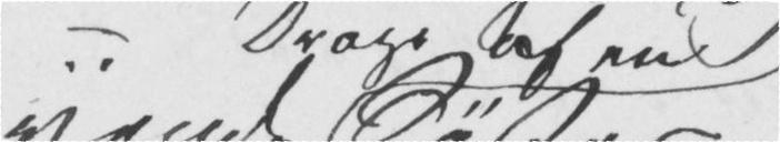Drage af en
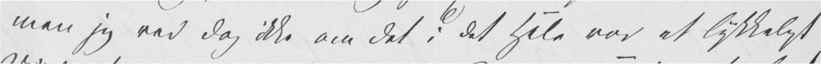men jeg ved dog ikke om det i det Hele var et lykkeligt
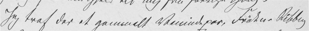Jeg traf der et gammelt Venindepar, Frøken Ribbing
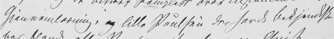Gjennemlæsning, og lille Paulsen der havde bekjendtska-
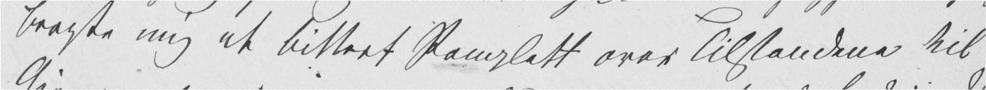bragte mig at bittert Pamplett over Tilstandene til
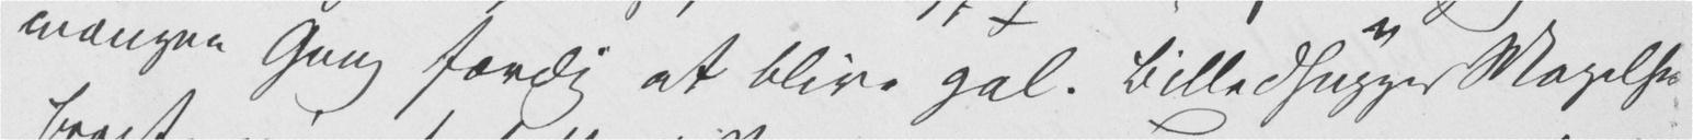mangen Gang færdig at blive gal. Billedhugger Magelse
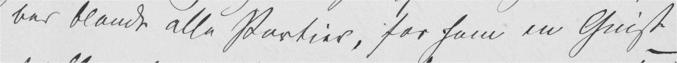ber blandt alle Partier, for som en Gnist
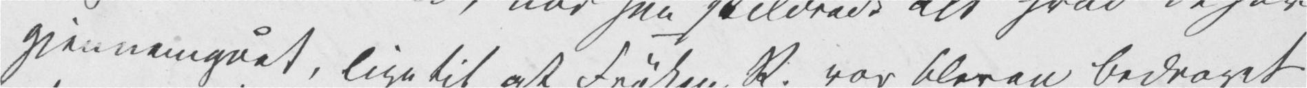gjennemgået, lige til at Frøken R. var bleven bedraget
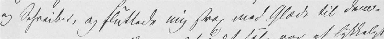og Schreiber, og sluttede mig strax med Glæde til dem.
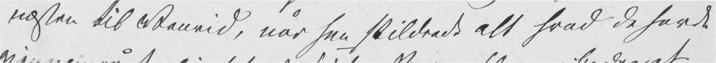næsten til Vanvid, når hun skildrede alt hvad de havde
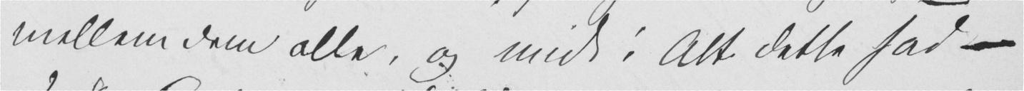mellem dem alle, og midt i Alt dette sad –
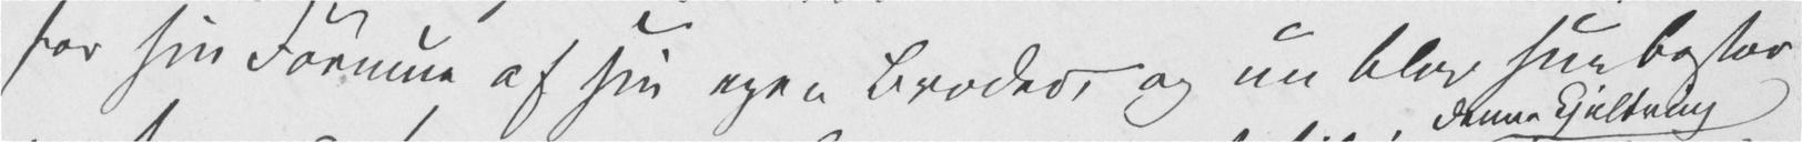for sin Formue af sin egen Broder, og nu blev hun bestor
